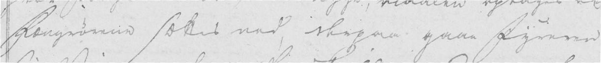Fængrørene sættes ned, derpaa gaar Fyreren
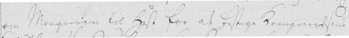om Morgenen til Hest for at bestige Kronprindsens
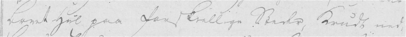lavet Hul paa forskiellige Stede, Krudt ned-
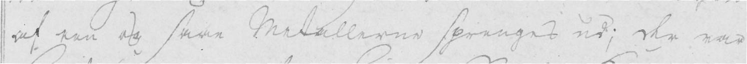af een og saae Metallerne sprenges ud; der var
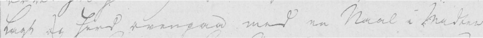lagt og Jord ovenpaa med en Naal i Midten
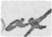af
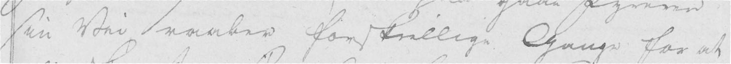sin Vei, raaber forskellige Gange for at
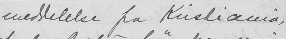meddelelse fra Kristiania,
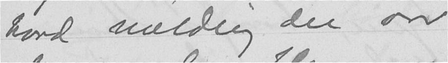hvad melding der var
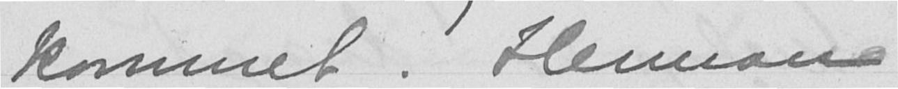kommet. Herman
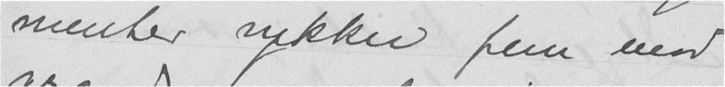menter rykker frem mod
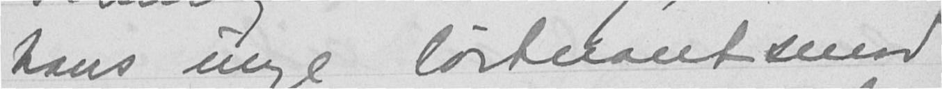hans unge løitnantsmod
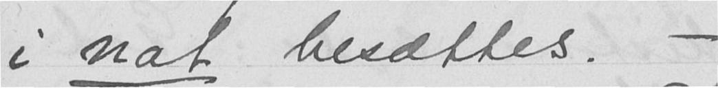i nat besættes. -
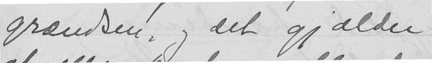grændsen, og det gjælder
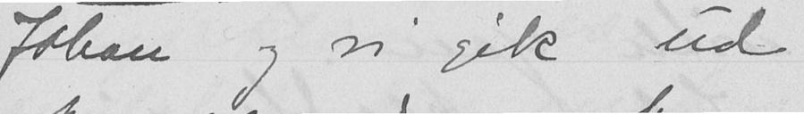Johan og vi gik ud
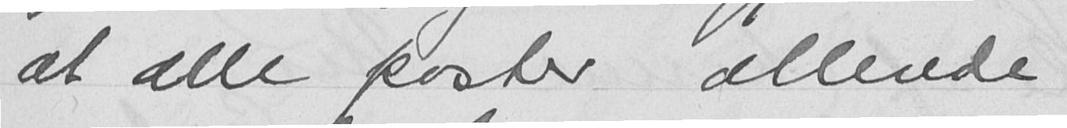at alle poster allerede
